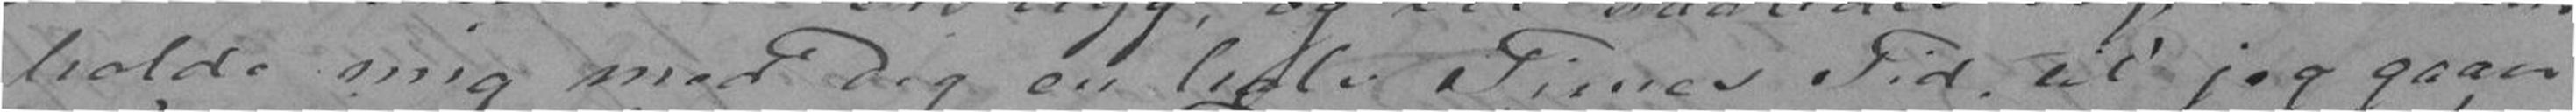holde mig med Dig en halv Times Tid til jeg gaaer
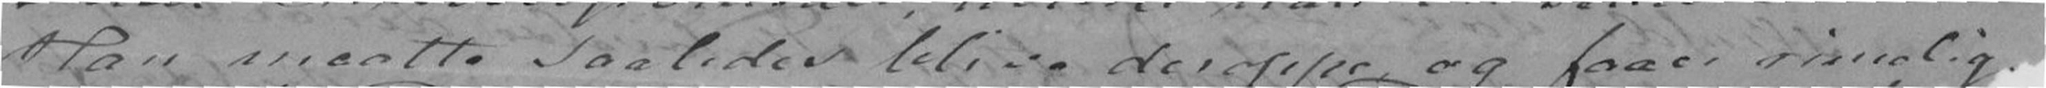Han maatte saaledes blive deroppe, og faaer rimelig-
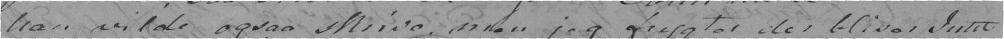han vilde ogsaa skrive, men jeg frygter der bliver Intet
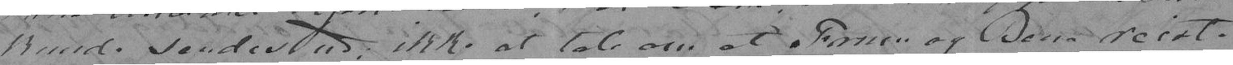kunde sendes ud, ikke at tale om at Fruen og Bene reiste
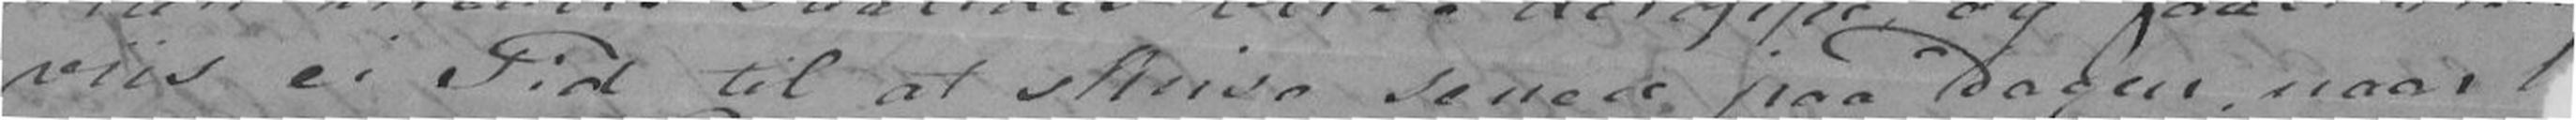viis ei Tid til at skrive senere paa Dagen, naar
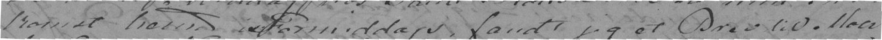komst herned i Formiddags fandt jeg et Brev til Moer
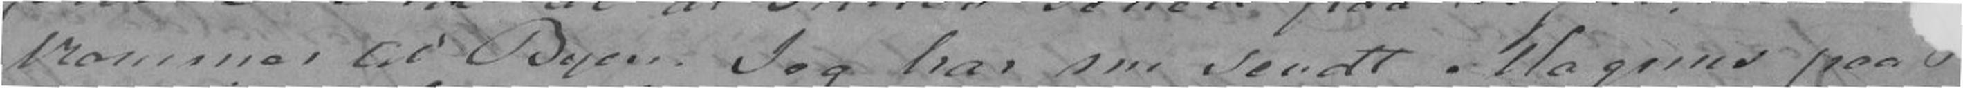kommer til Byen. Jeg har nu sendt Magnus paa
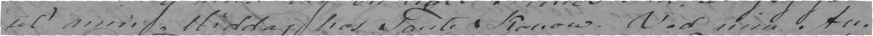til min Middag hos Tante Konow. Ved min An-
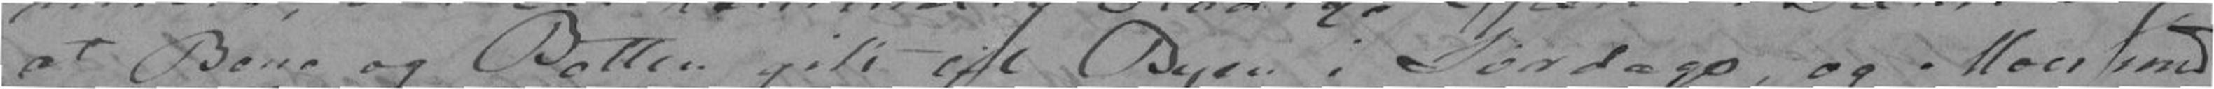at Bene og Betten gik til Byen i lørdags, og Moer med
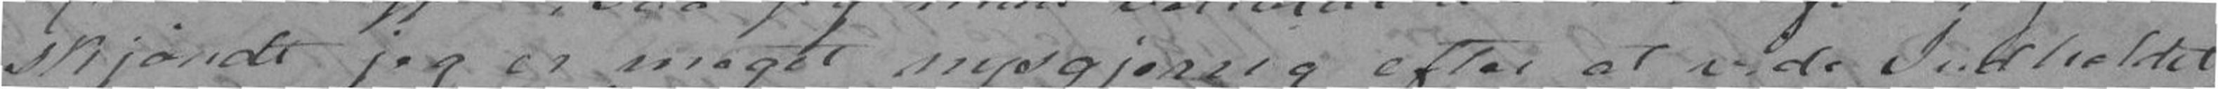skjøndt jeg er meget nysgjerrig efter at vide Indholdet,
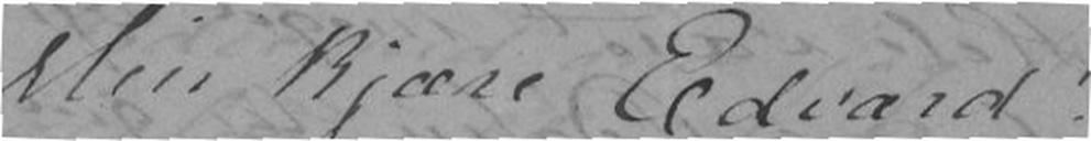Min kjære Edvard!
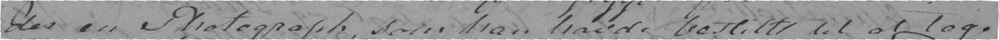der en Photograph, som han havde bestilt til at tage
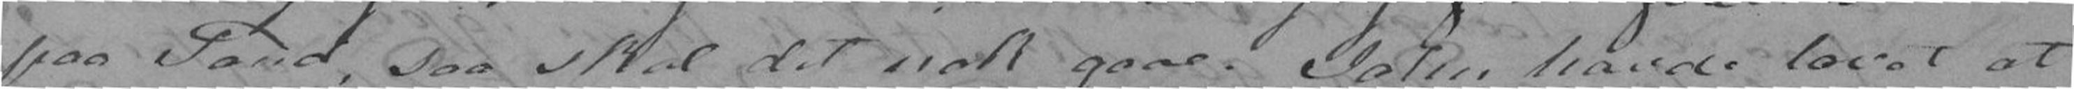paa Tand, saa skal det nok gaae. John havde lovet at
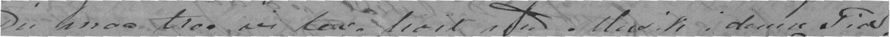Du maa troe vi leve høit med Musik i denne Tid,
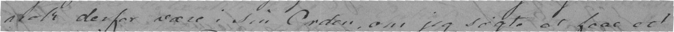nok derfor være i sin Orden, om jeg søgte at faae et
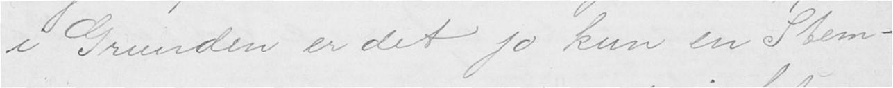i Grunden er det jo kun en Stem-
